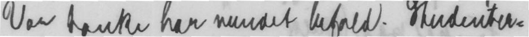Vor tanke har vundet bifald. Studenter-
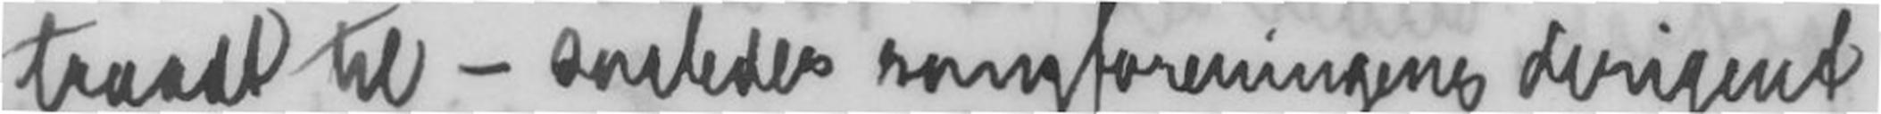traadt til - saaledes sangforeningens dirigent
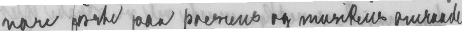vore første paa poesiens og musikens omraade,
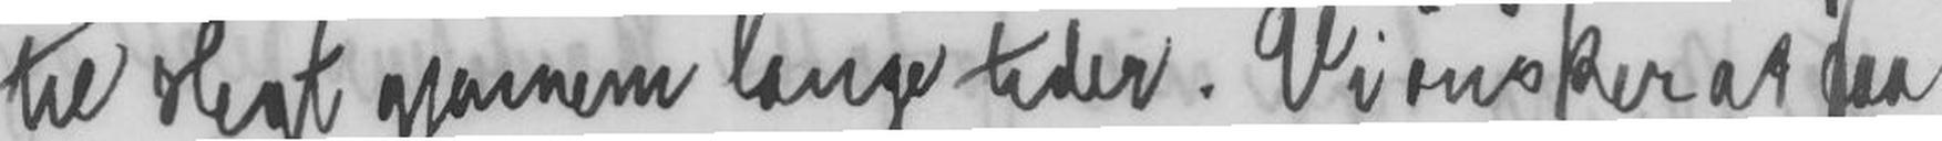til slegt gjennem lange tider. Vi ønsker at faa
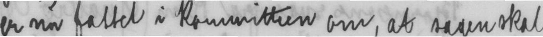er nu fattet i kommitteen om, at sagen skal
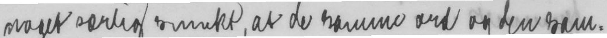noget særlig smukt, at de samme ord og den sam-
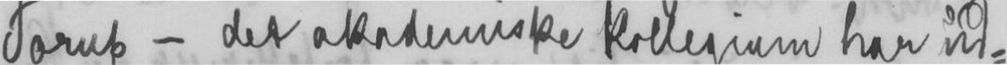Torup - det akademiske kollegium har ud-
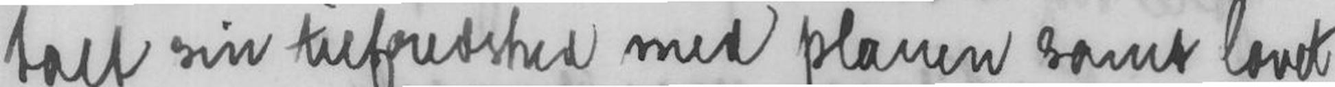talt sin tilfredshed med planen samt lovet
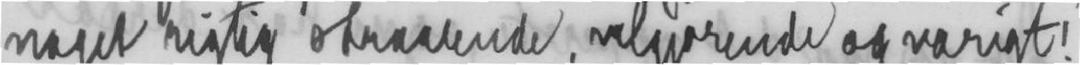noget rigtig straalende, velgjørende og varigt!
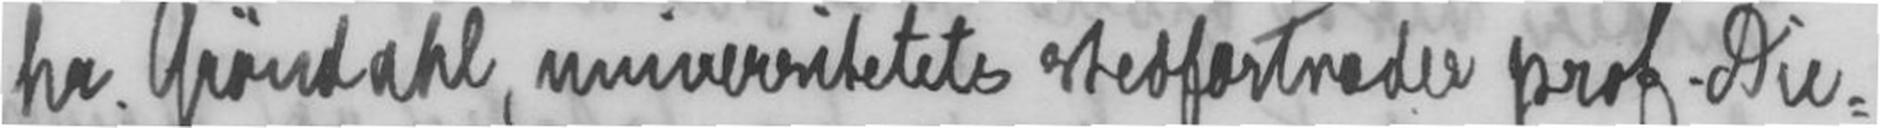hr. Grøndahl, universitetets stedfortræder prof. Die-
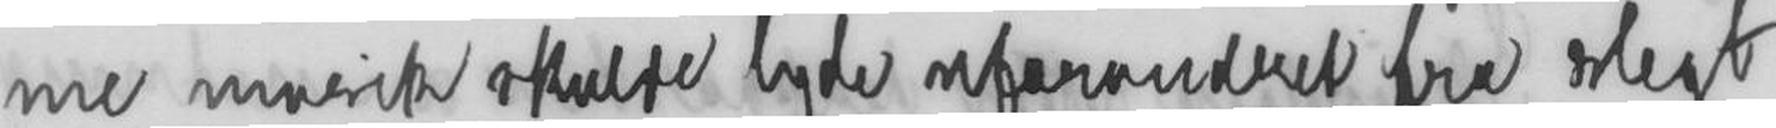me musik skulde lyde uforandret fra slegt
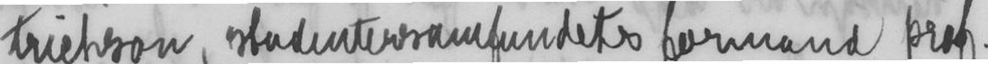trichson, studentetsamfundets formand prof.
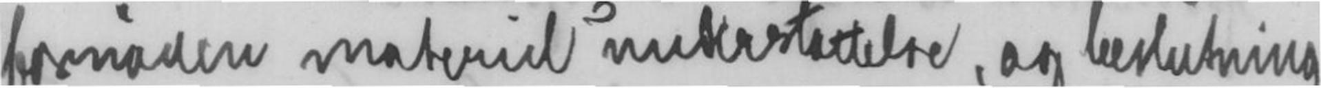fornøden materiel understøttelse, og beslutning
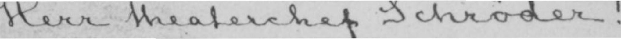Herr theaterchef Schrøder!
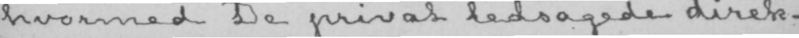hvormed De privat ledsagede
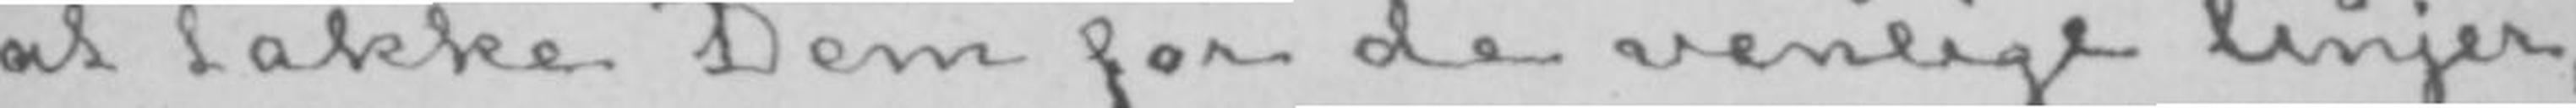at takke Dem for de venlige linjer,
